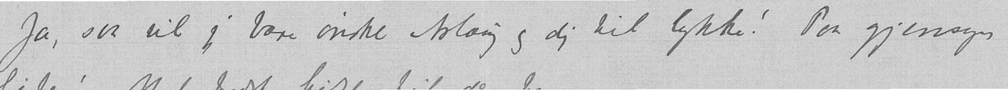Ja, saa vil jeg bare ønske Aslaug og dig til lykke! Paa gjensyn
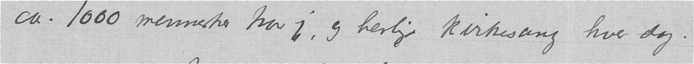ca. 1000 mennesker tror jeg, og herlige kirkesang hver dag.
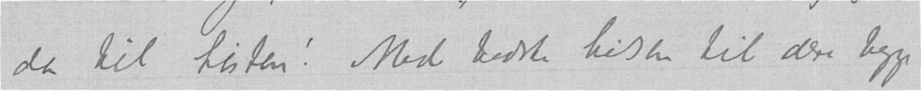da til høsten! Med bedste hilsen til dere begge
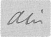din
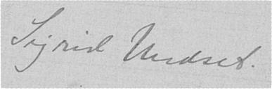Sigrid Undset.
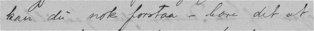kan du nok forstaa — bare det at
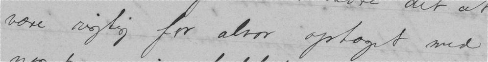være rigtig for alvor optaget med
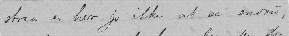straa er her jo ikke at se endnu,
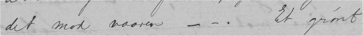det mod vaaren — —. Et grønt
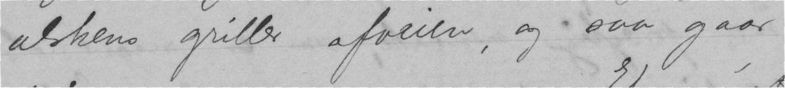alskens griller afveien, og saa gaar
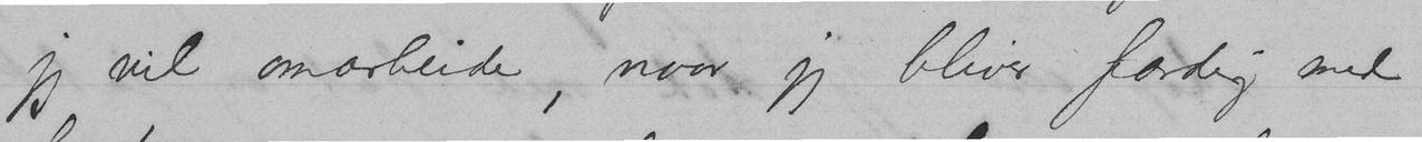jeg vil omarbeide, naar jeg bliver færdig med
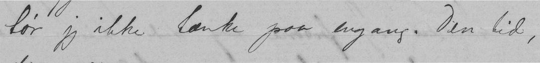tør jeg ikke tænke paa engang. Den tid,
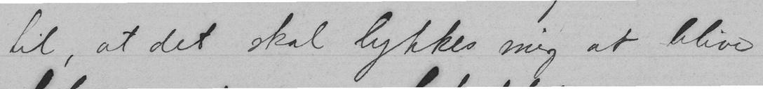til, at det skal lykkes mig at blive
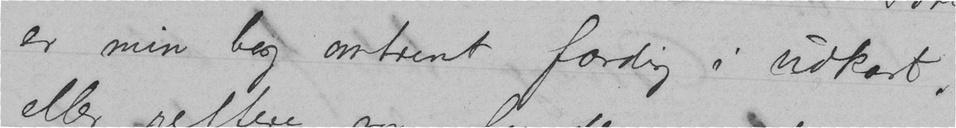er min bog omtrent færdig i udkast,
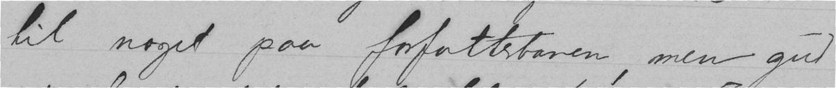til noget paa forfatterbanen, men gud
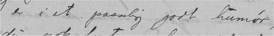Jeg er i et passelig godt humør,
